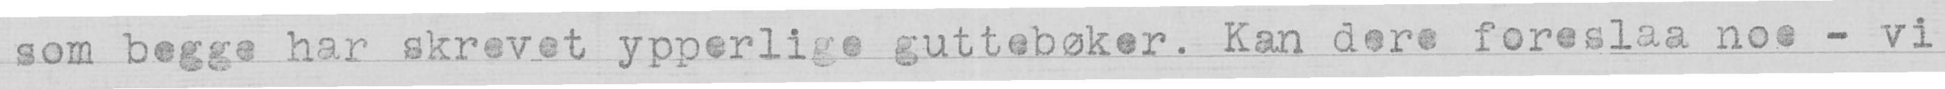som begge har skrevet ypperlige guttebøker. Kan dere foreslaa noe - vi
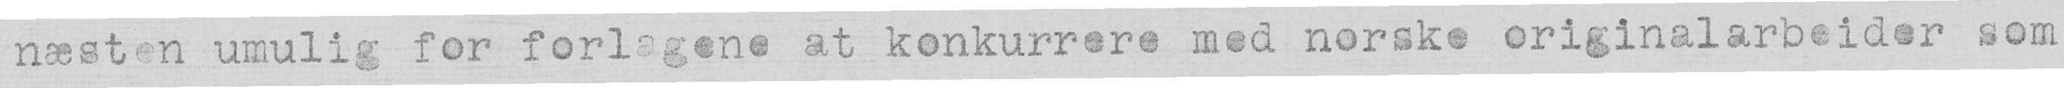næsten umulig for forlagene at konkurrere med norske originalarbeider som
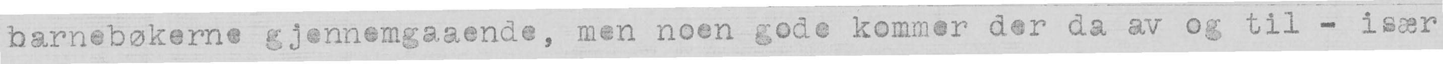barnebøkerne gjennemgaaende, men noen gode kommer der da av og til - iser
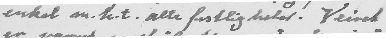enkel m.h.t. alle festligheter. Veiret
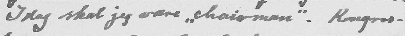Idag skal jeg være "chairman". Kongres-
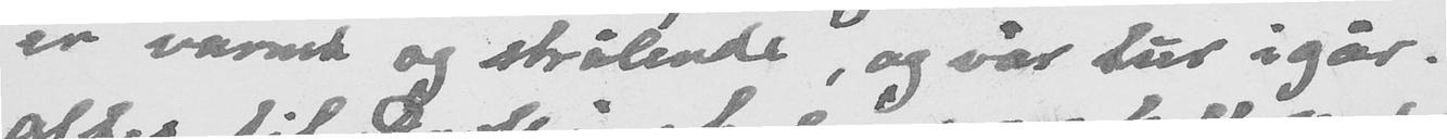er varmt og strålende, og vår tur i går
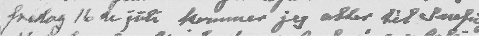fredag 16de juli kommer jeg atter til Snefugl.
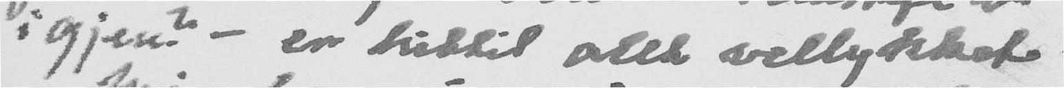igjen? - er hittil allt vellykket.
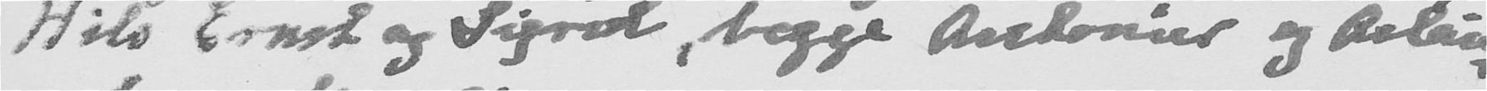Hils Ernst og Sigrid, begge Antonier og Erling
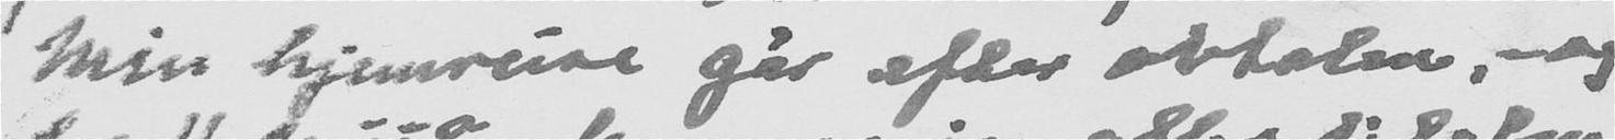Min hjemreise går efter avtalen, og
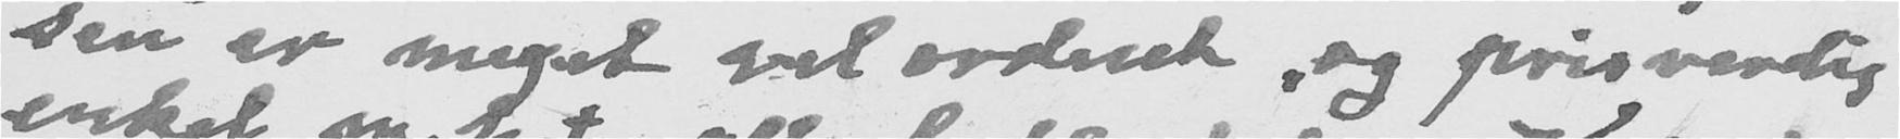sen er meget vel ordnet, og prisverdig
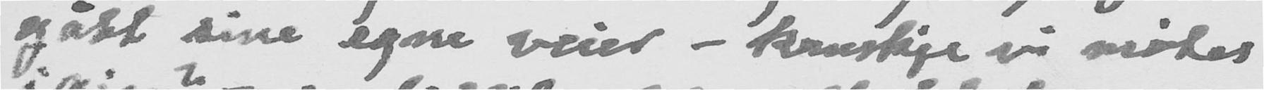gått sine egne veier - kanskje vi møtes
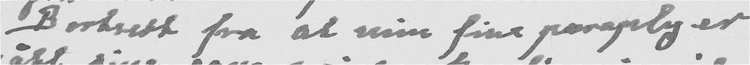Bortsett fra at min fine paraply er
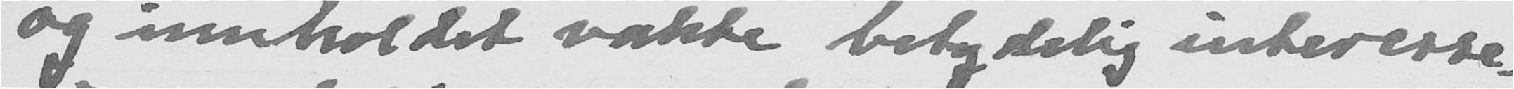og innholdet vakte betydelig interesse.
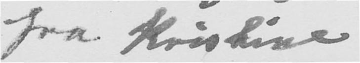fra Kristine
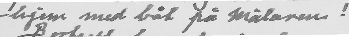- hjem med båt på Mälaren!
